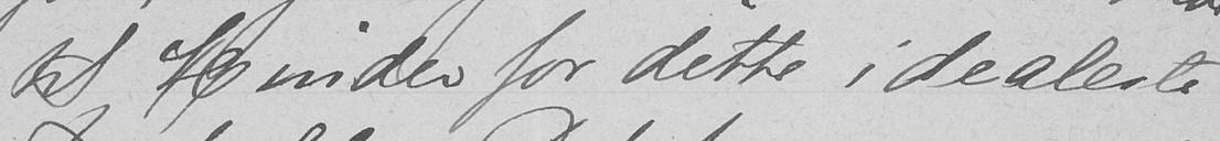til Hinder for dette idealeste
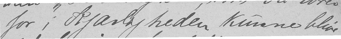for i Kjærligheden kunne blive
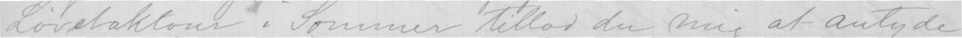Løvstaktour i Sommer tillod du mig at antyde
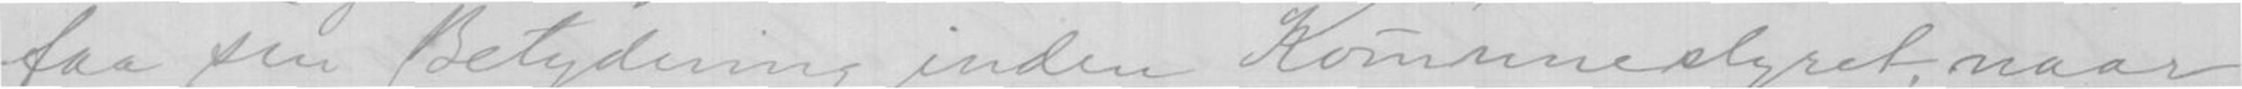faa sin Betydning inden Komunestyret, naar
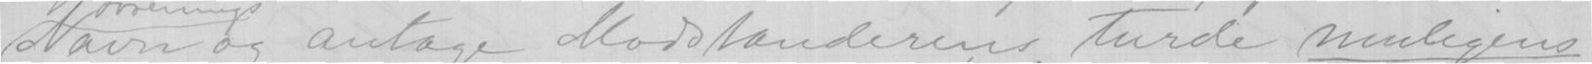Navn og antage Modstanderens, turde muligens
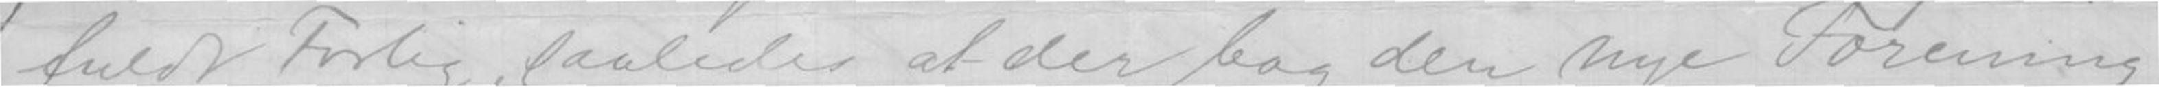fuldt Forlig, saaledes at der bag den nye Forening
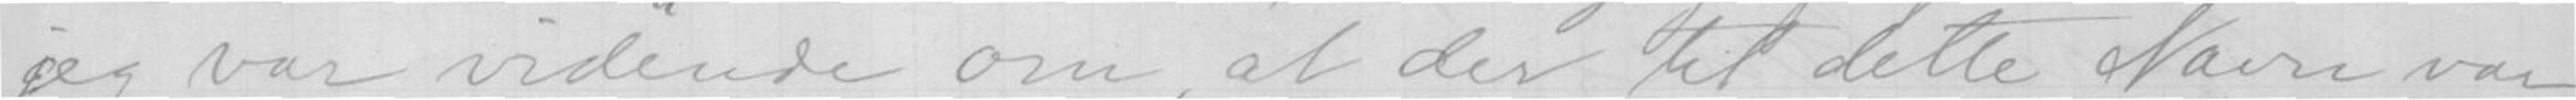jeg var vidende om, at der til dette Navn var
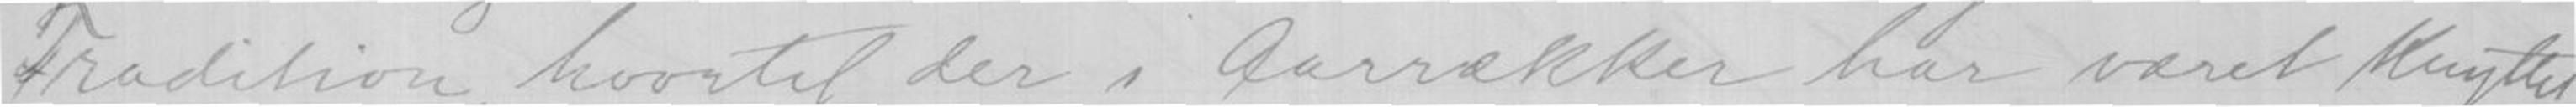Tradition, hvortil der i Aarrækker har været knyttet
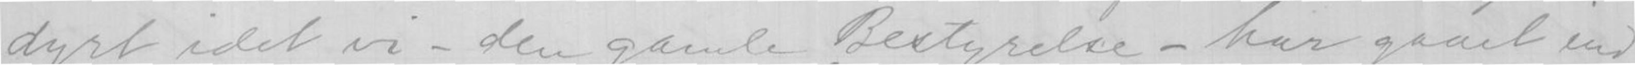dyrt idet vi - den gamle Bestyrelse - har gaaet ind
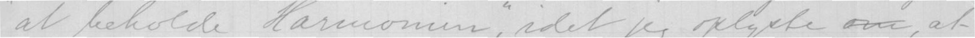at beholde Harmonien, idet jeg oplyste om, at
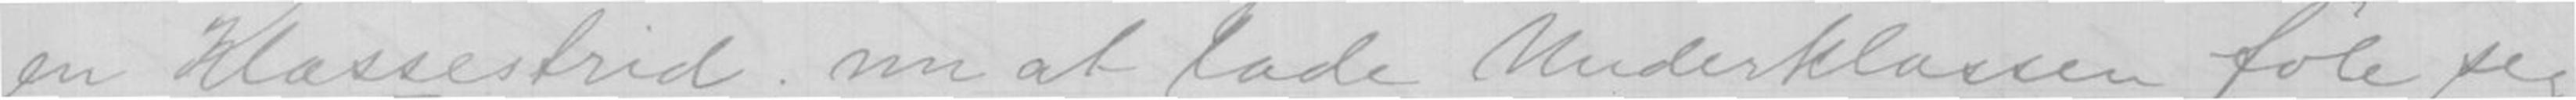en Klassestrid; nu at lade Underklassen føle sig
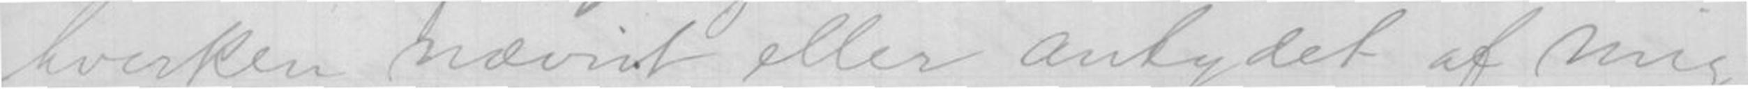hverken nævnt eller antydet af mig.
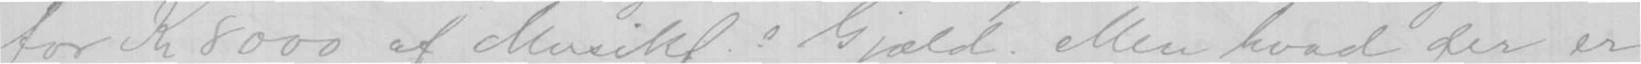for Kr 8000 af Musikf.s Gjæld. Men hvad der er
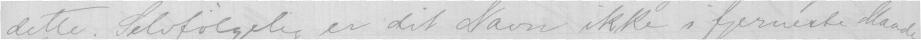dette. Selvfølgelig er dit Navn ikke i fjerneste Maade
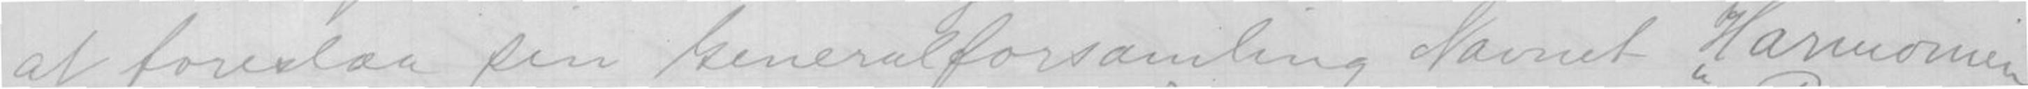at foreslaa sin Generalforsamling Navnet Harmonien,
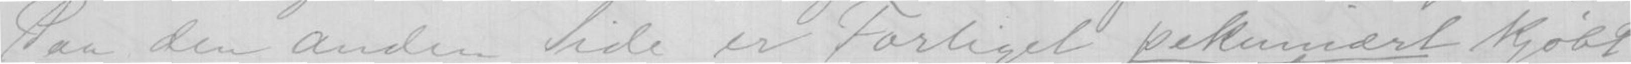Paa den anden Side er Forliget pekuniært kjøbt
